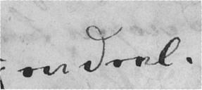en deel.
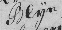Blye
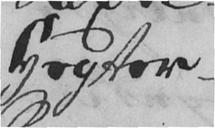Hegter
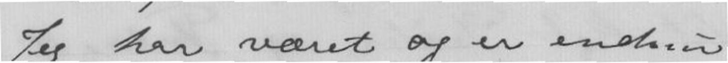Jeg har været og er endnu
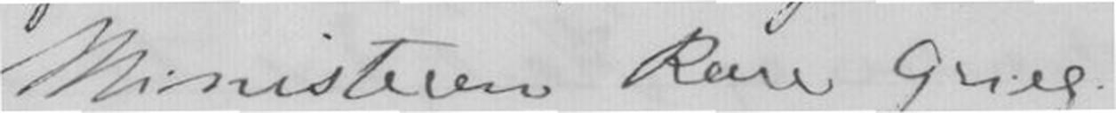Ministeren Kære Grieg.
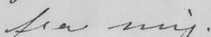fra mig.
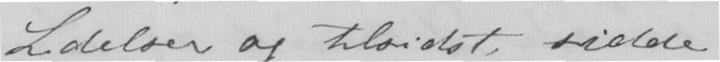Lidelser og tilsidst, sidde
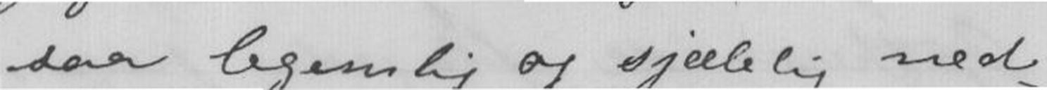saa legemlig og sjelelig ned-
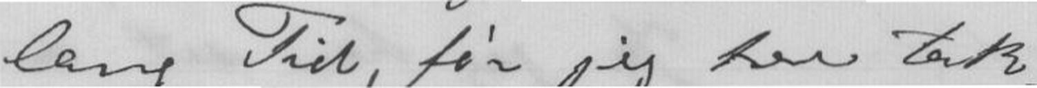lang Tid, før jeg har tak-
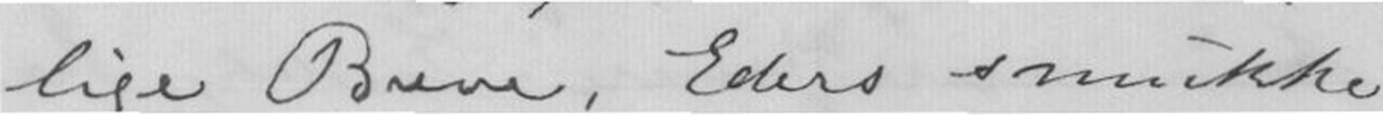lige Breve, Eders smukke
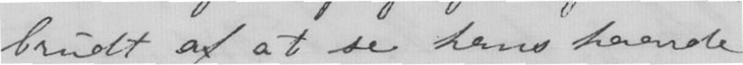brudt af at se hans haarde
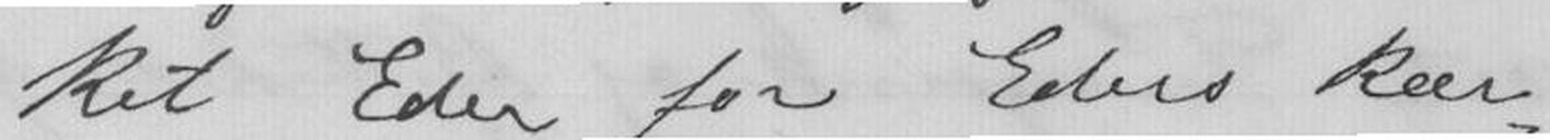ket Eder for Eders kær-
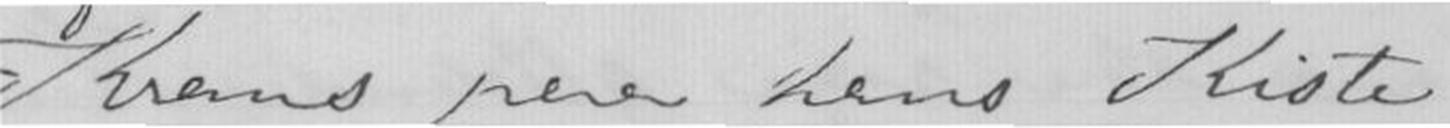Krans paa hans Kiste
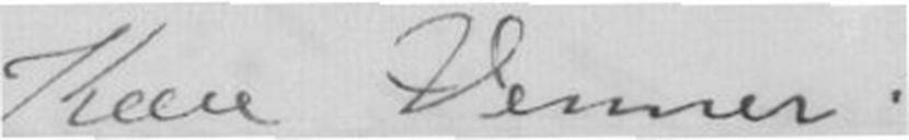Kære Venner!
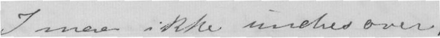I maa ikke undres over,
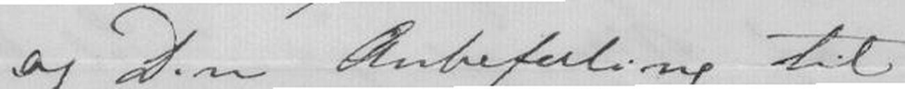og Din Anbefaling til
